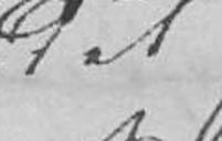Det
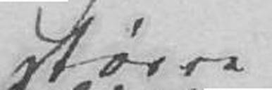større
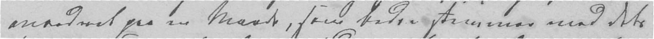anordnet paa en Maade, som bedre stemmer med dets
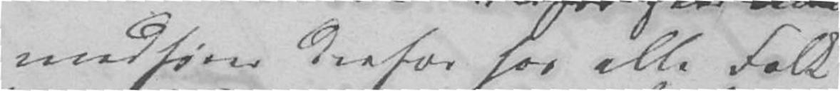medfører derfor for alle Folk
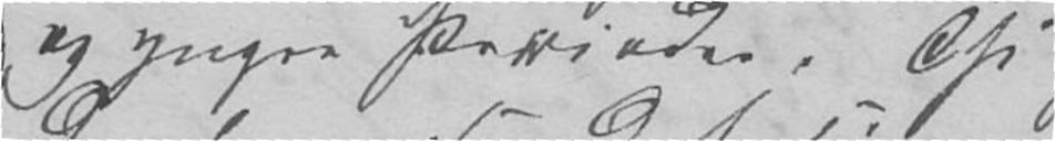og yngre Periode. Thi
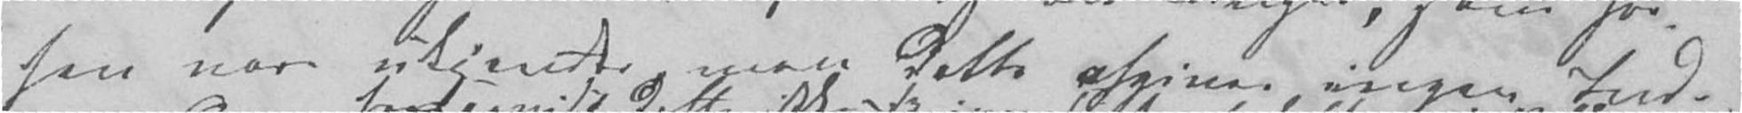hen var ukjendte, men dette afgiver ingen Ind-
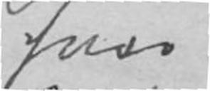hvor
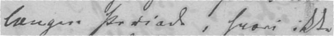længere Periode, hvori ikke
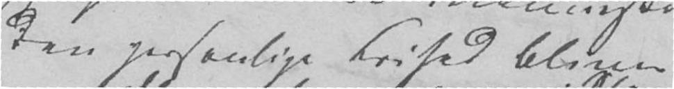Den personlige Frihed bliver
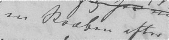en Stræben efter
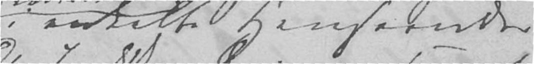i enkelte Henseender
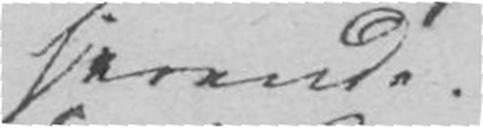serende.
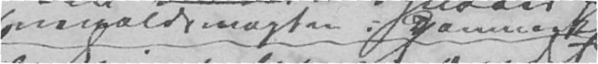Enevoldsmagten i Danmark
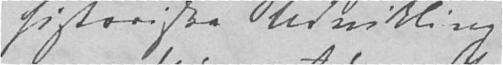historiske Udvikling
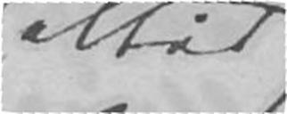altid
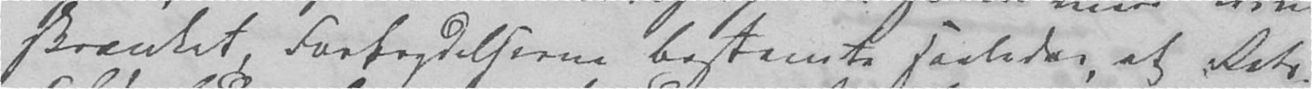skrænket, Forbrydelserne bestemte saaledes, at Rets-
